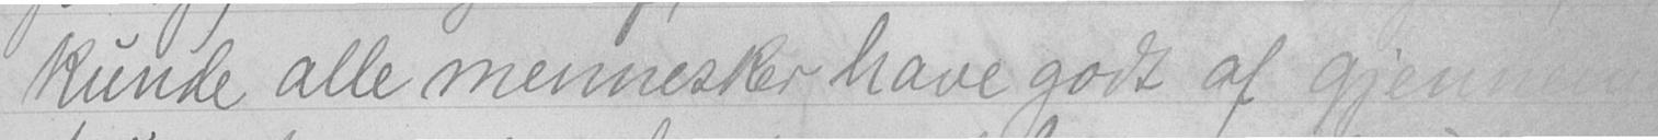kunde alle mennesker have godt af gjennem
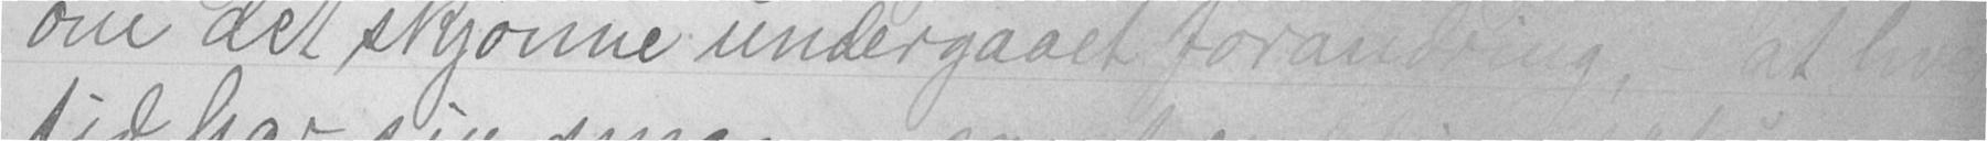om det skjønne undergaaet forandring, at hver
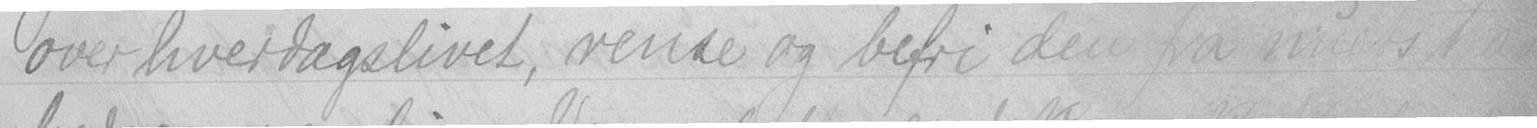over hveddagslivet, rense og befri den fra nuets travel-
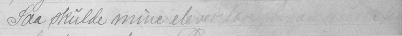Saa skulde mine elever lære, hvad kunsten
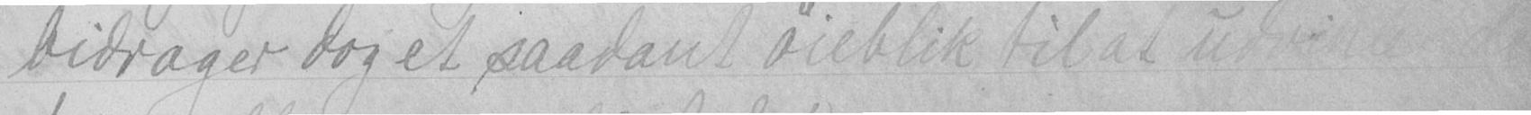bidrager dog et saadant øieblik til at udvikle den
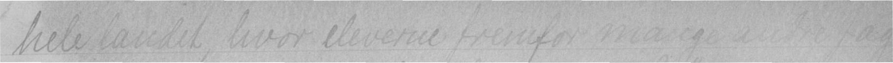hele landet, hvor eleverne fremfor mange andre fag
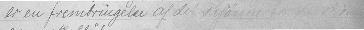er en frembringelse af det skjønne for det skjøndes
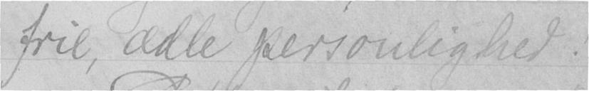frie, adle personlighed!
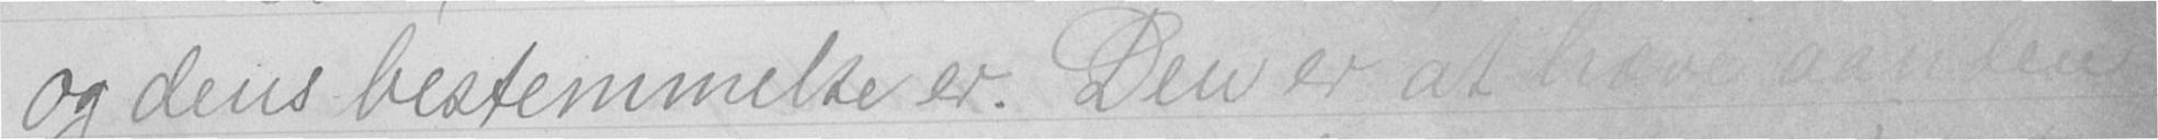og dens bestemmelse er. Den er at hæve aanden
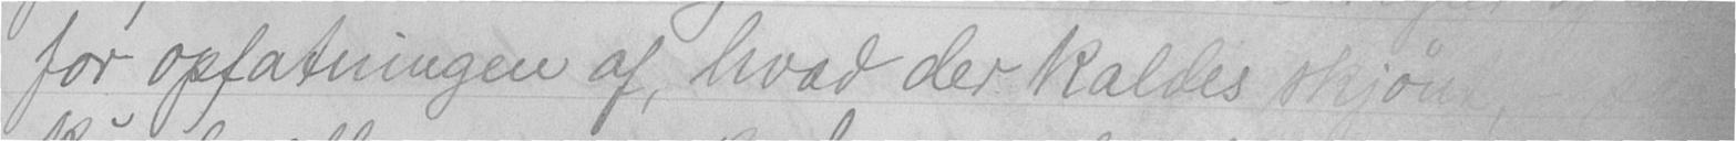for opfatningen af, hvad der kaldes skjønt, saa
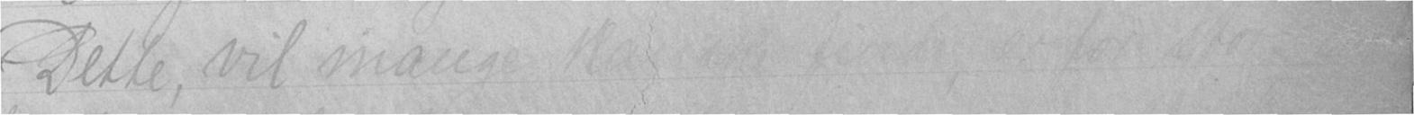Dette, vil mange kanske finde, er for stort
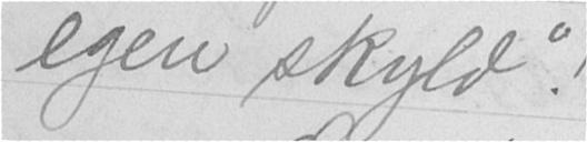egen skyld"!
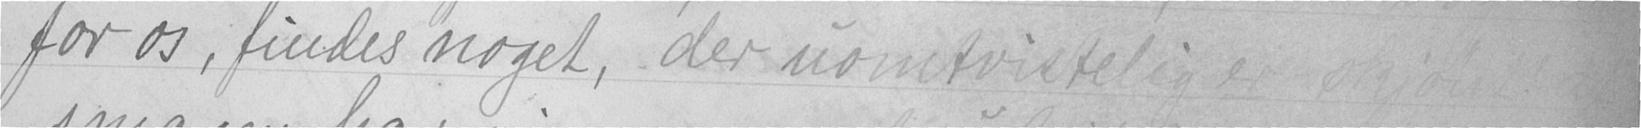for os, findes noget, der uomtvistelig er skjønt! at
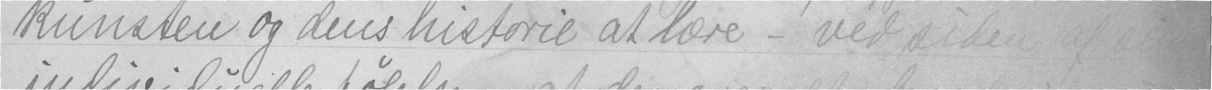kunsten og dens historie at lære - ved siden af sin
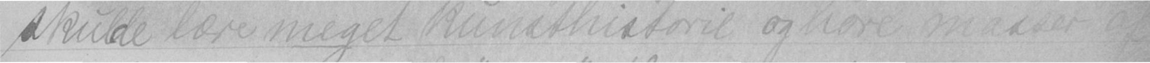skulle lære meget kunsthistorie og høre masser af
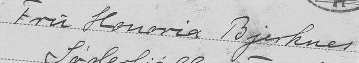Fru Honoria Bjerknes
